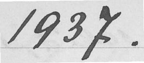1937.
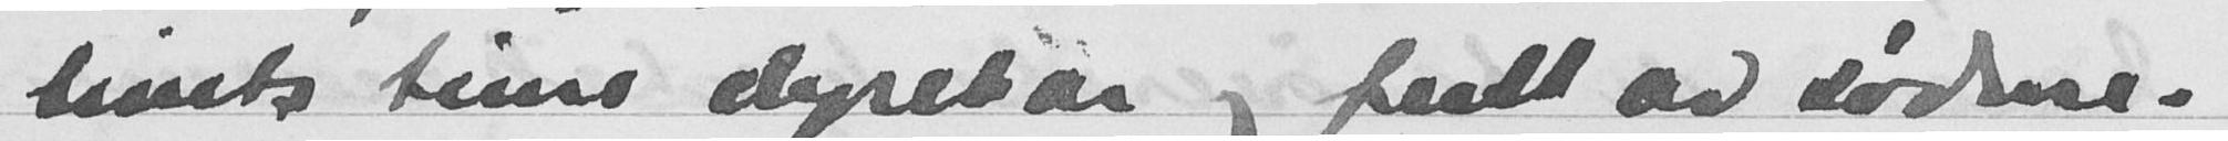turets tims dyretar og trett av døden.
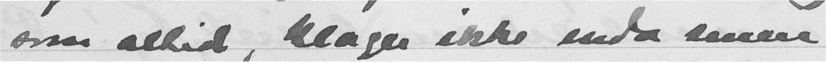som altid, klager ikke enda sneen
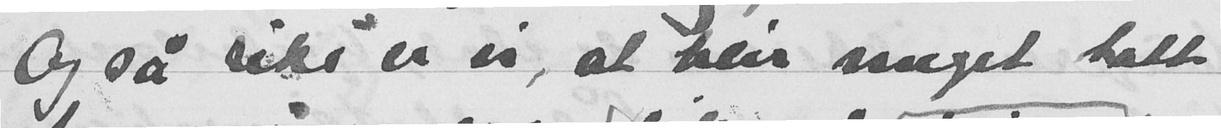Og så rike er vi, at blir meget tatt
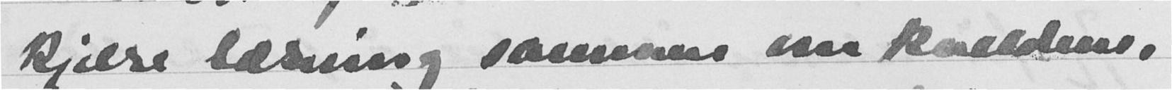kjære læsning sammen om kvelden,
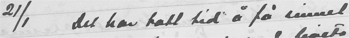21/1 Det har tatt tid i få sinnet
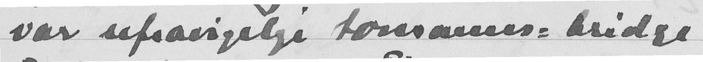vår ufravigelige tomanns-bridge
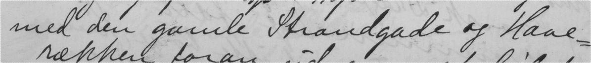med den gamle Strandgade og Have-
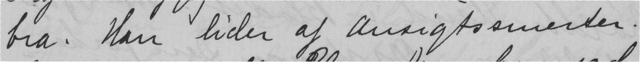bra. Han lider af Ansigtssmerter.
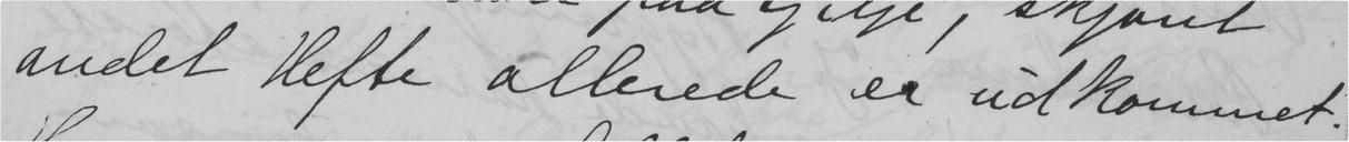andet Hefte allerede er udkommet.
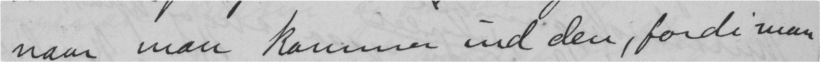naar man kommer ind der, fordi man
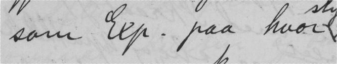som Exp. paa hvor
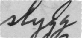stygg
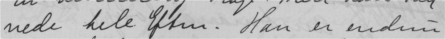nede hele Efterm. Han er endnu
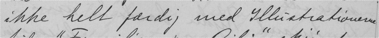ikke helt færdig med Illustrationerne
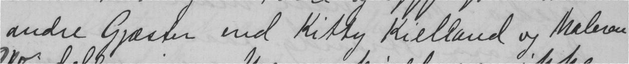andre Gjæster end Kitty Kielland og Maleren
